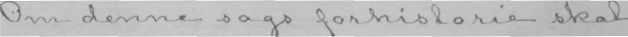Om denne sags forhistorie skal
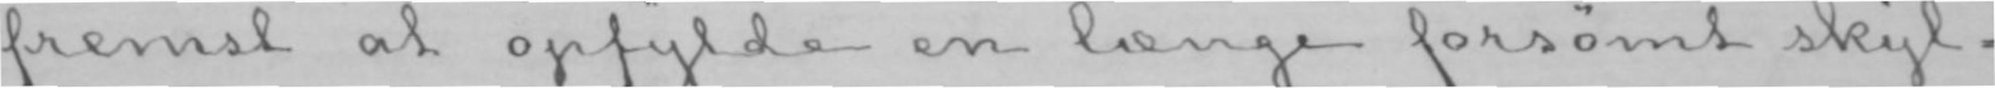fremst at opfylde en længe forsømt skyl-
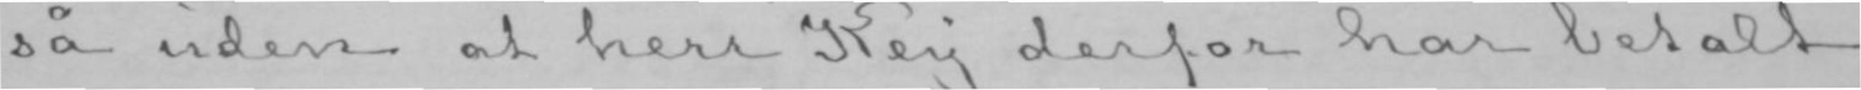så uden at herr Key derfor har betalt
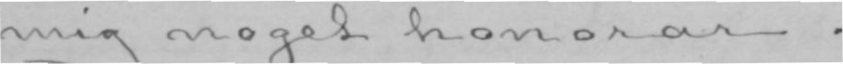mig noget honorar.
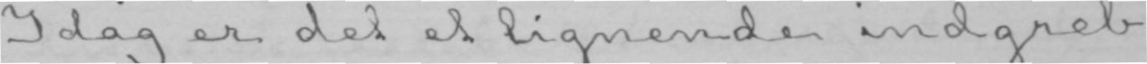Idag er det et lignende indgreb
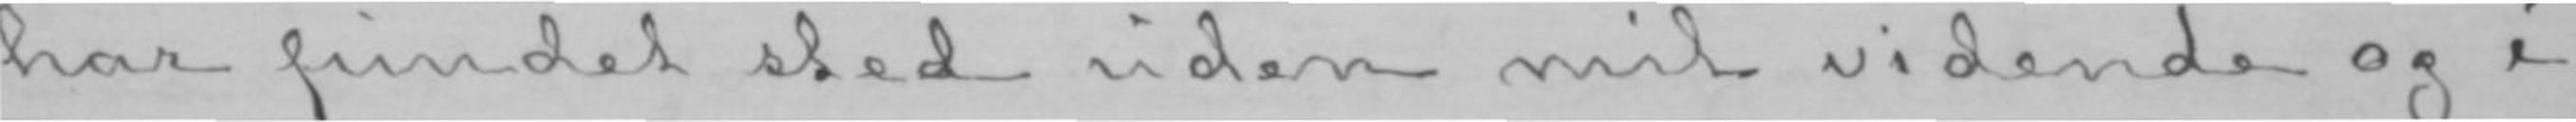har fundet sted uden mit vidende og i-
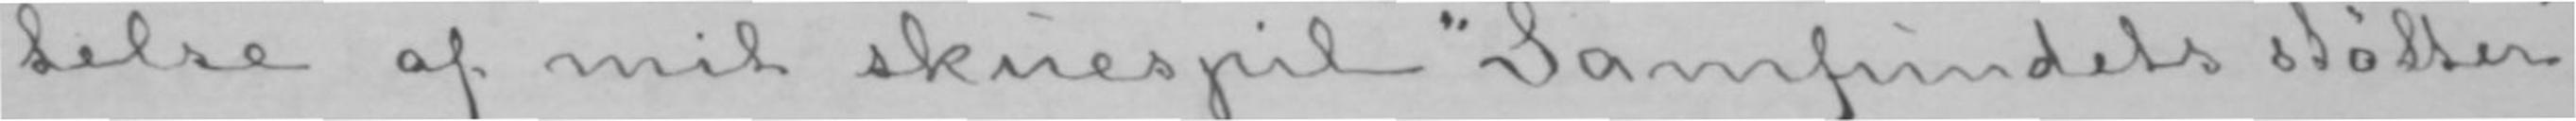telse af mit skuespil «Samfundets støtter»
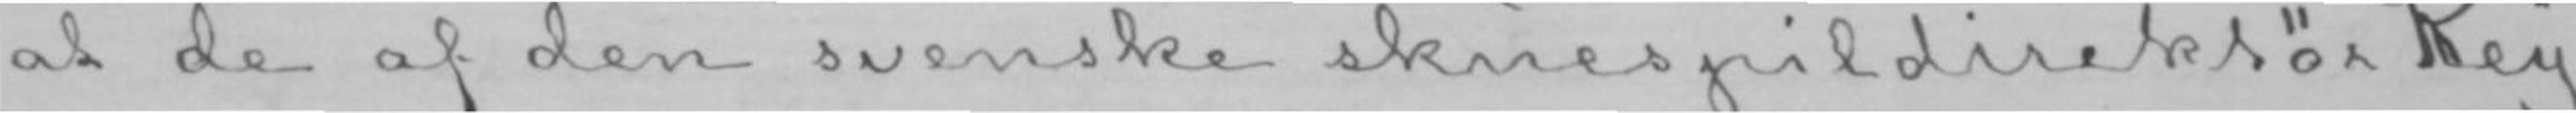at de af den svenske skuespildirektør Key
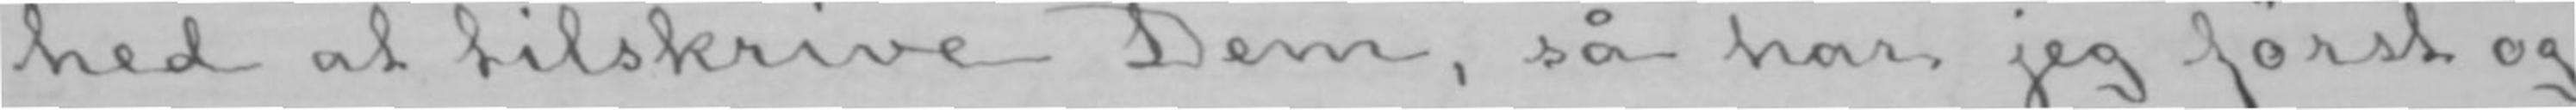hed at tilskrive Dem, så har jeg først og
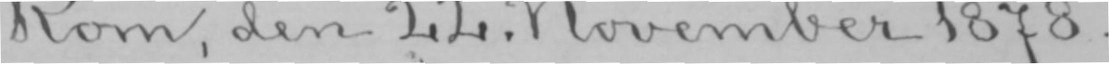Rom, den 22. November 1878.
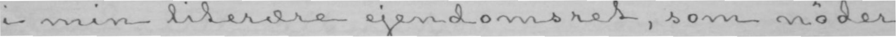i min literære ejendomsret, som nøder
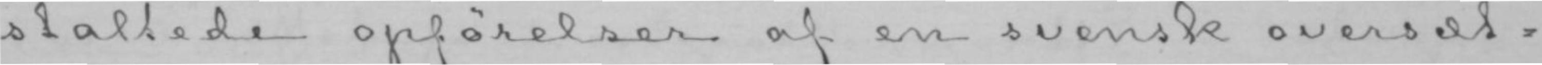staltede opførelser af en svensk oversæt-
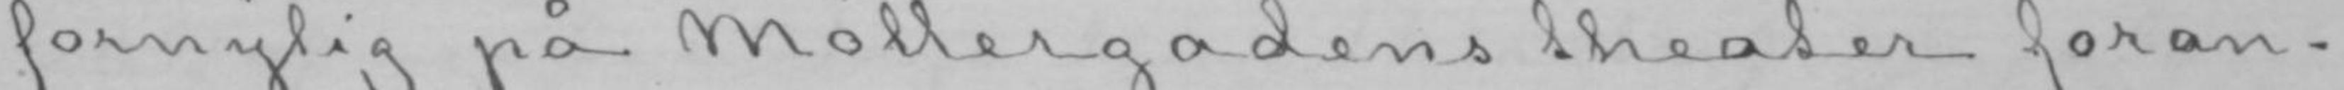fornylig på Møllergadens theater foran-
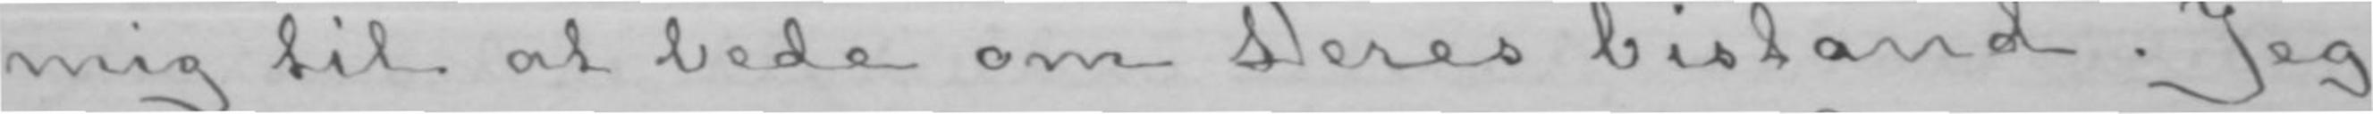mig til at bede om Deres bistand. Jeg
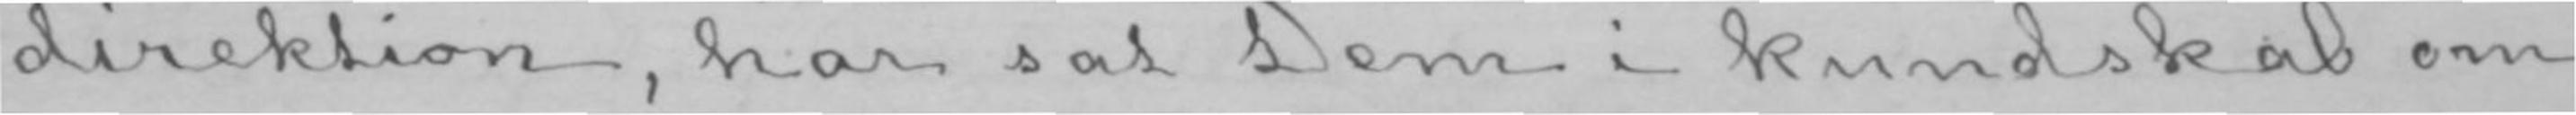direktion, har sat Dem i kundskab om
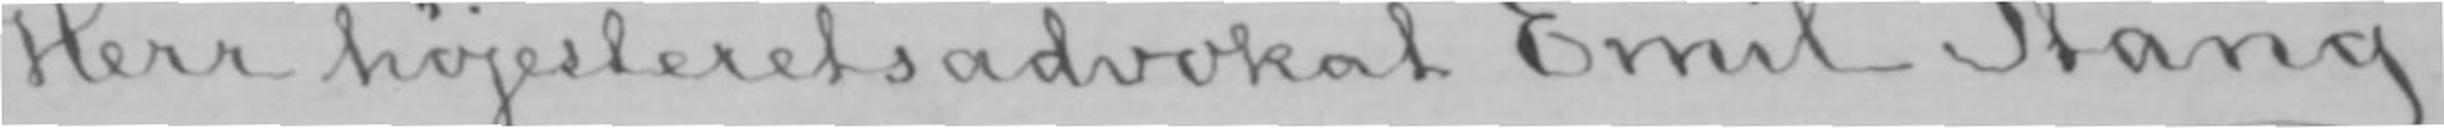Herr højesteretsadvokat Emil Stang!
